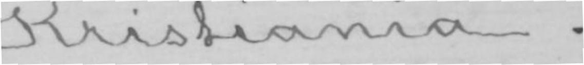Kristiania.
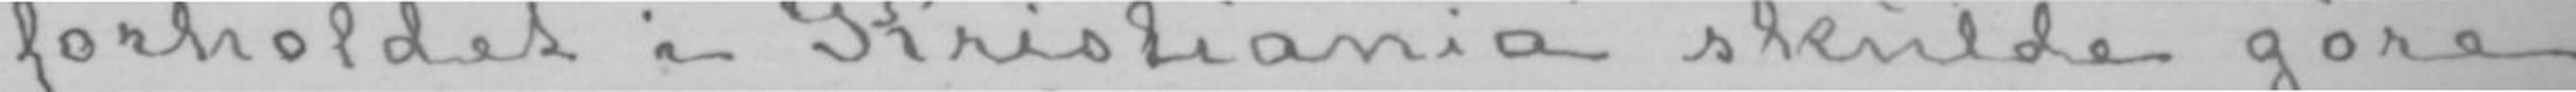forholdet i Kristiania skulde gøre
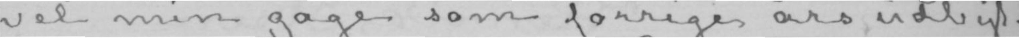vel min gage som forrige års udbyt-
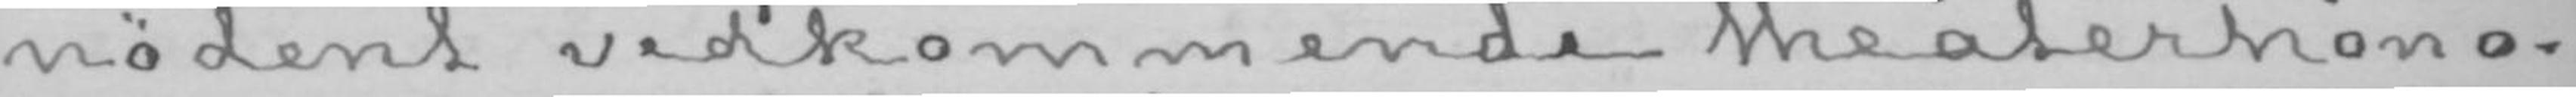nødent vedkommende theaterhono-
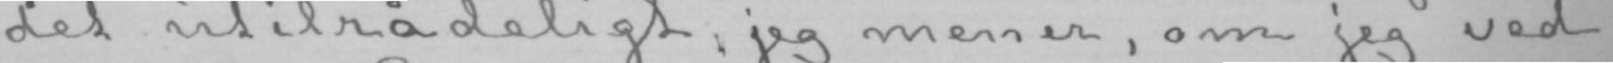det utilrådeligt; jeg mener, om jeg ved
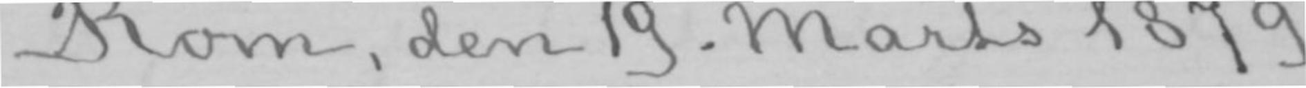Rom, den 19. Marts 1879.
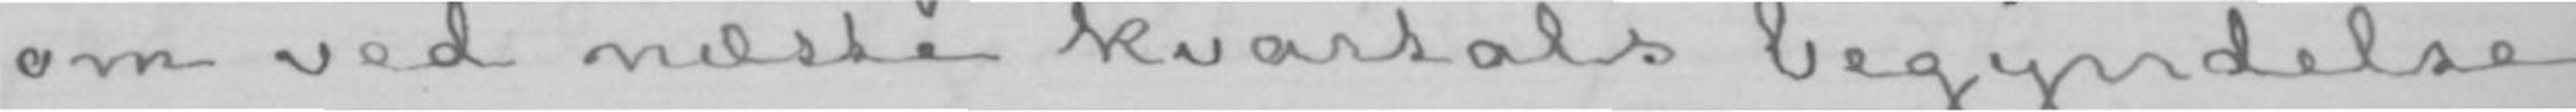om ved næste kvartals begyndelse,
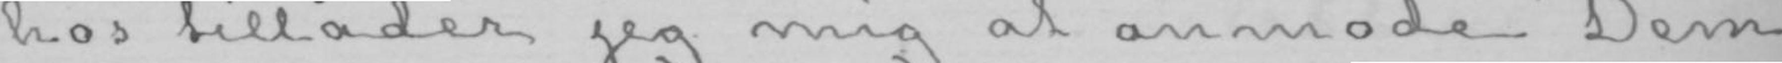hos tillader jeg mig at anmode Dem
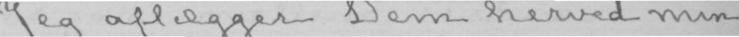Jeg aflægger Dem herved min
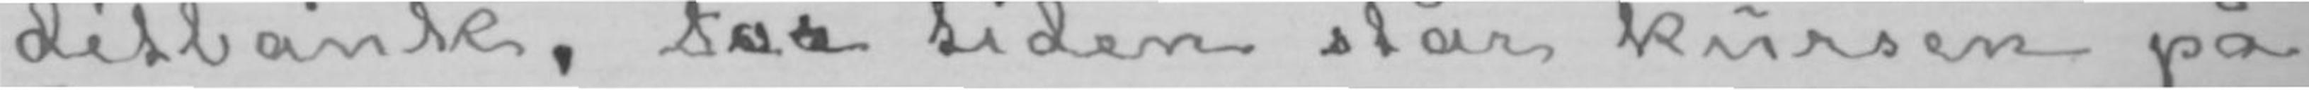ditbank. For tiden står kursen på
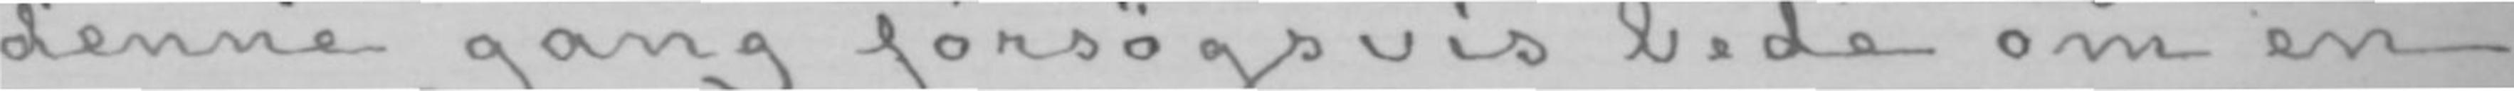denne gang forsøgsvis bede om en
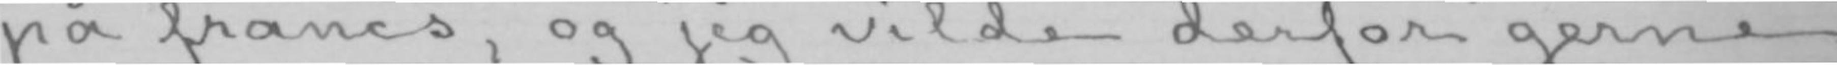på francs, og jeg vilde derfor gerne
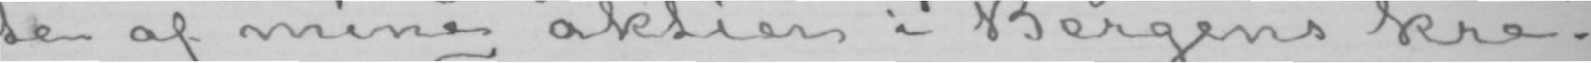te af mine aktier i Bergens kre-
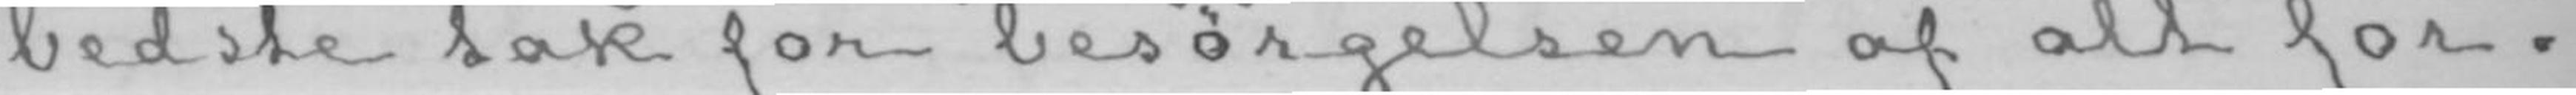bedste tak for besørgelsen af alt for-
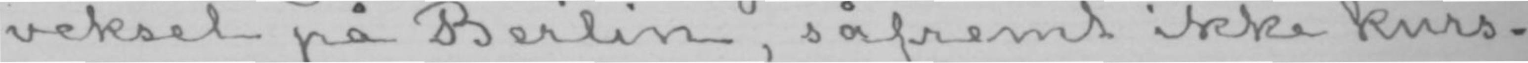veksel på Berlin, såfremt ikke kurs-
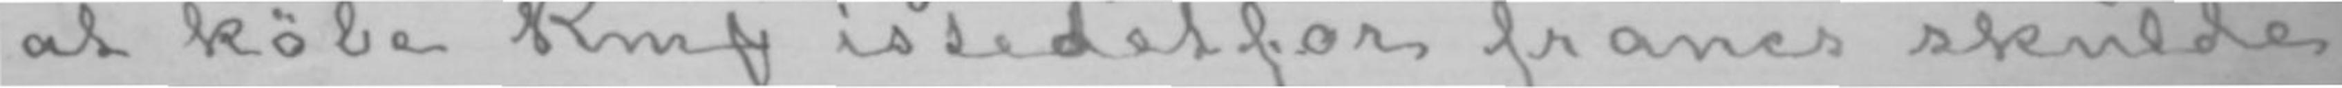at købe RmՖ istedetfor francs skulde
